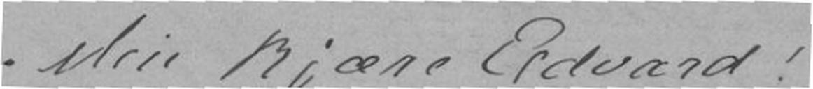Min kjære Edvard!
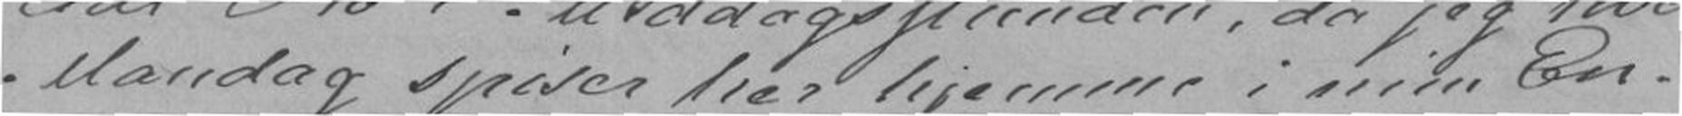Mandag spiser her hjemme i min En-
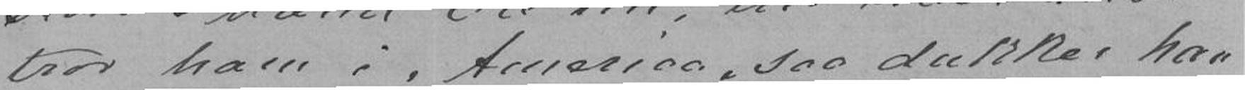tror ham i America, saa dukker han
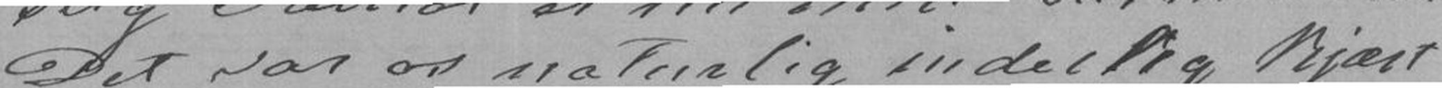Det var os naturlig inderlig kjært
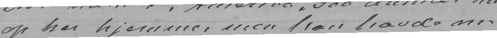op her hjemme, men han havde nu
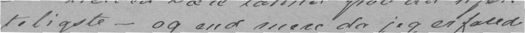teligste - og end mere da jeg erfarede
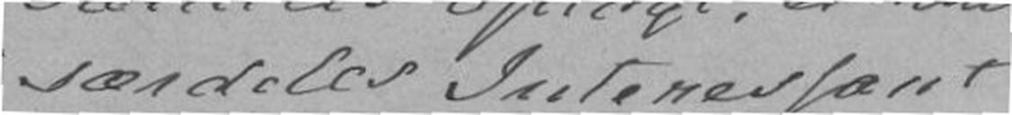særdeles Interessant
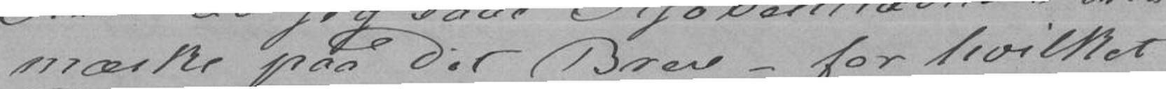mærke paa Dit Brev - for hvilket
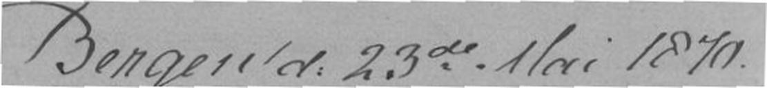Bergen d. 23de Mai 1870.
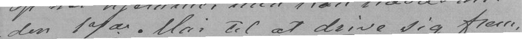den 17de Mai til at drive sig frem,
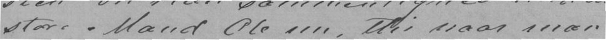store Mand Ole nu, thi naar man
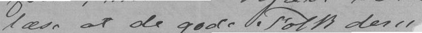læse at de gode Folk dern
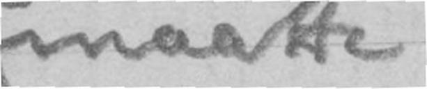maatte
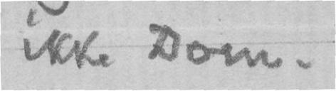ikke Dom.
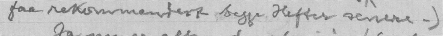faa rekommandert begge Hefter senere.)
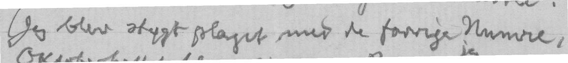(Jeg blev stygt plaget med de forrige Numre,
